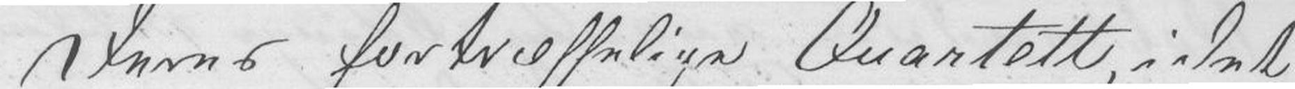Deres fortræffelige Quartett i det
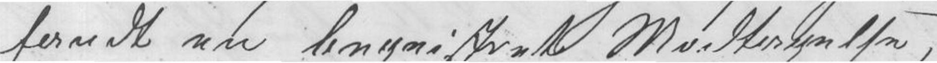fandt en begeistret Modtagelse,
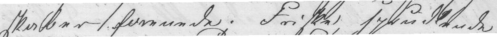skaber forenede. Friske sprudlende
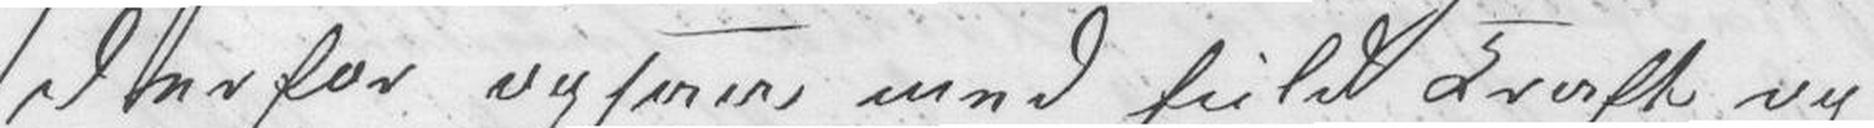derfor ogsaa med fuld Kraft og
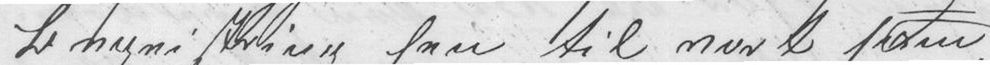Begeistring hen til vort sam-
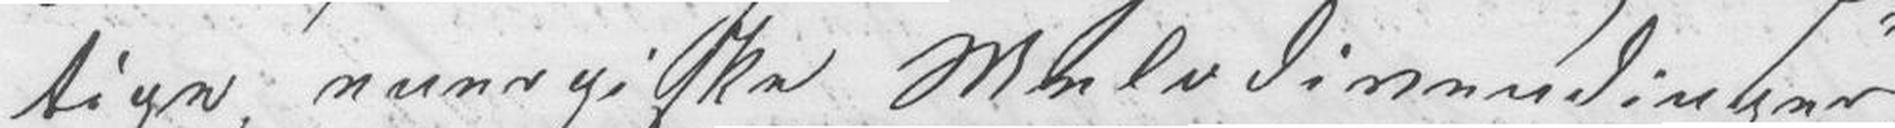tige energiske Melodivendinger,
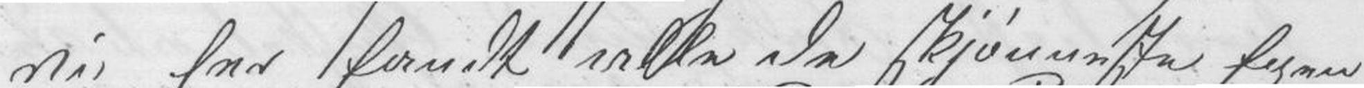vi her fandt alle de skjønneste Egen-
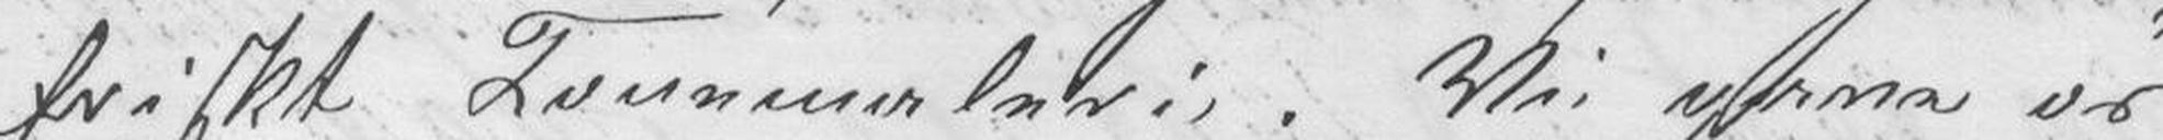friskt Tonemaleri. Vi gave os
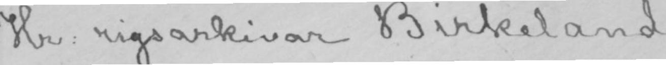Hr: rigsarkivar Birkeland.
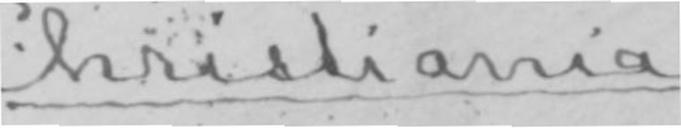Christiania
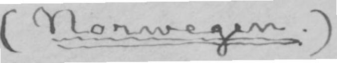(Norwegen.)
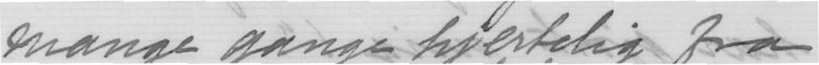mange ganger hjertelig fra
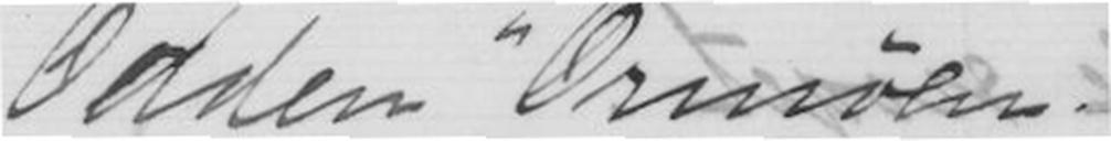"Odden"Ormøen.
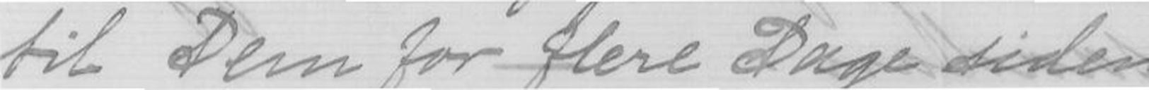til Dem for flere Dage siden,
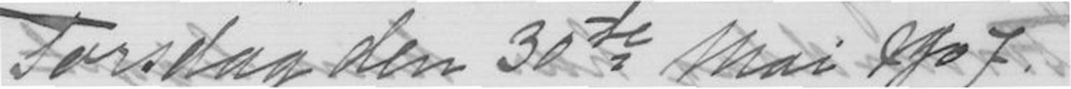Torsdag den 30te Mai 1907
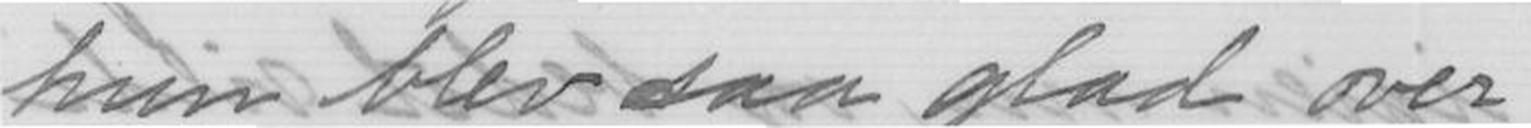hun blev saa glad over.
